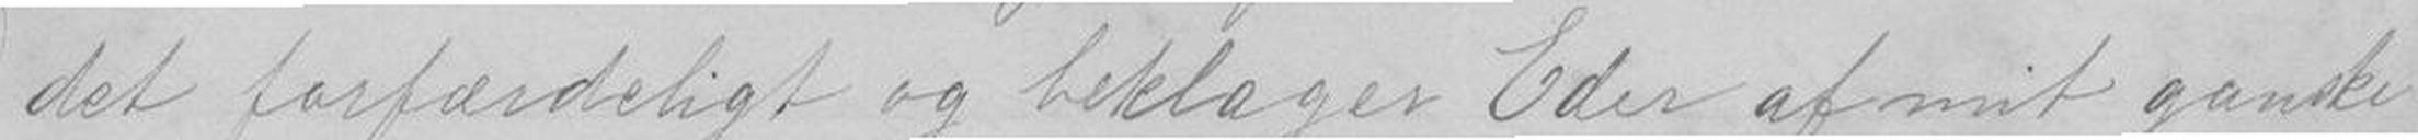det forfærdeligt og beklager Eder af mit ganske
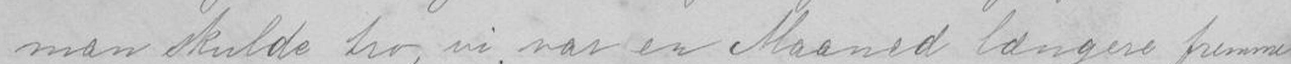man skulde tro, vi var en Maaned længere fremmere
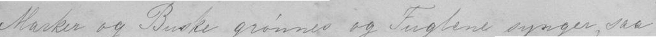Marker og Buske grønnes og Fuglene synger, saa
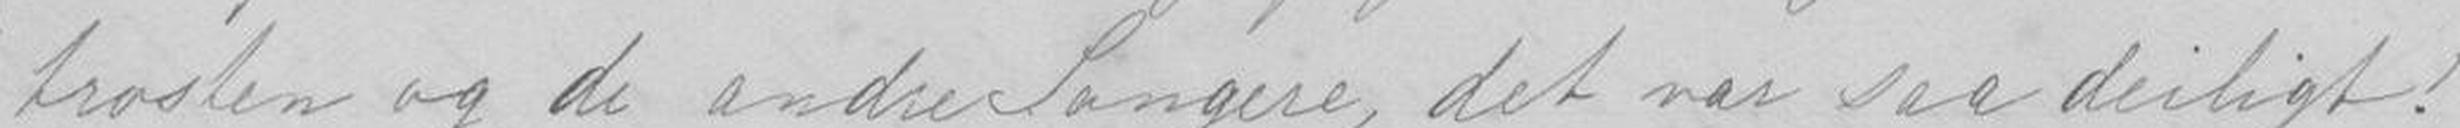trosten og de andre Sangere, det var saa deiligt!
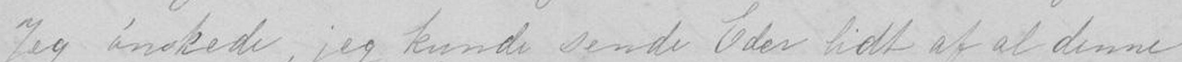Jeg Snakede, jeg kunde sende Eder Lidt af al denne
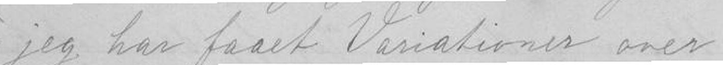jeg har faaet Variationer over
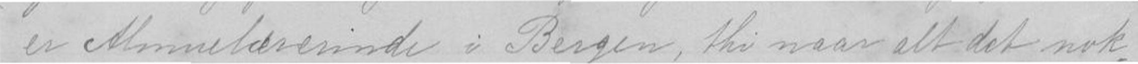er Almmelærerinde i Bergen, thi naar alt det nok-
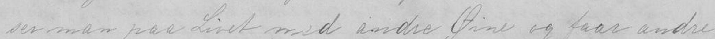ser man paa Livet med andre Øine og faar andre
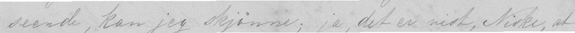seende, kan jeg skjønne; jo det er mest, Niske, at
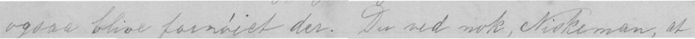ogsaa blive fornøiet der. Du ved nok, Niskeman, at
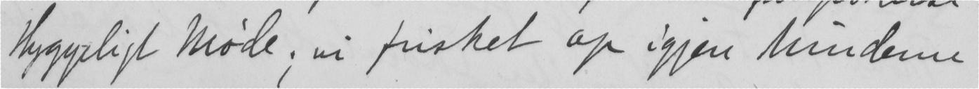Hyggeligt Møde; vi frisket op igjen Murderne
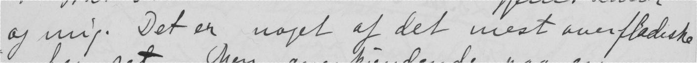og mig. Det er noget af det mest overfladiske
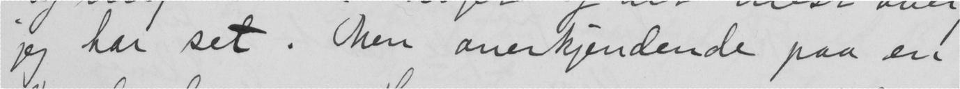jeg har set. Men anerkjendende paa en
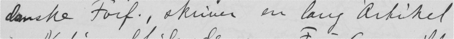danske Forf., skriver en lang Artikel
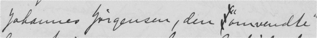Johannes Jørgensen, den "omvendte"
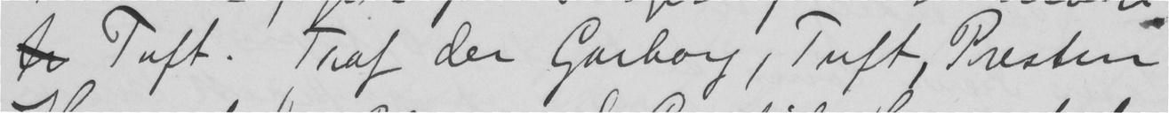Tuft. Traf der Garborg, Tuft, Presten
